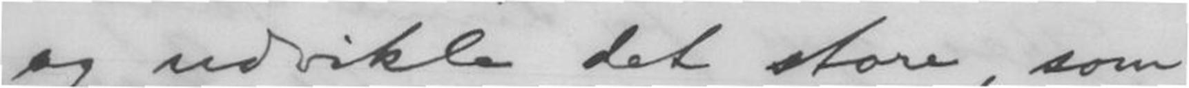og udvikle det store, som
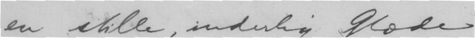en stille, inderlig Glæde
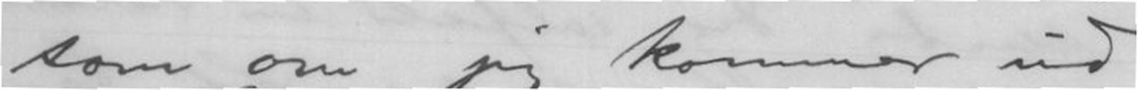som om jeg kommer ud
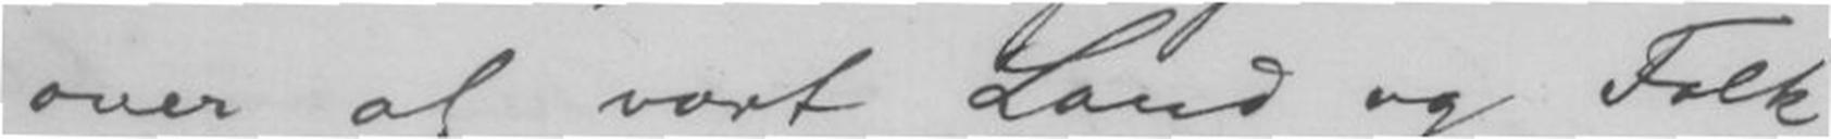over at vort Land og Folk
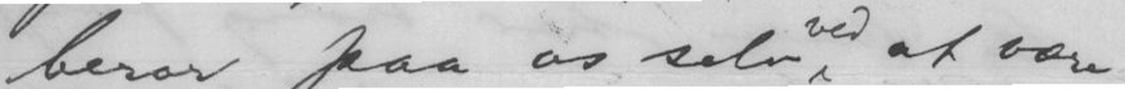beror paa os selv at være
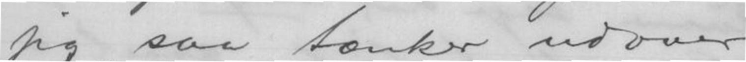jeg saa tænker udover
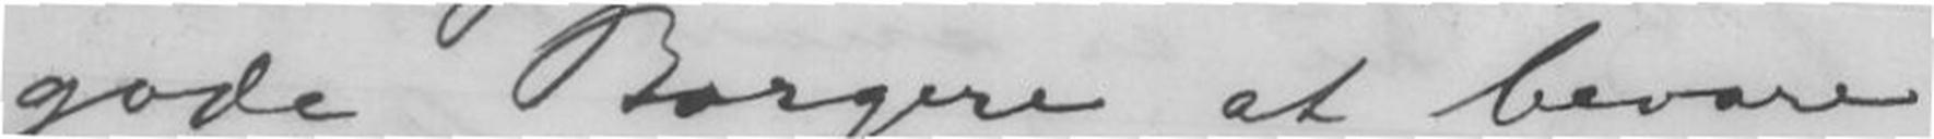gode Borgere at bevare
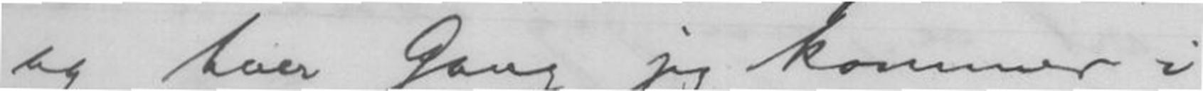og hver Gang jeg kommer i
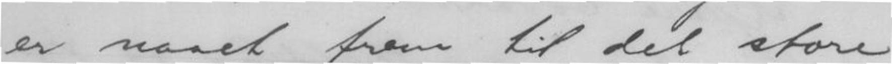er naaet frem til det store
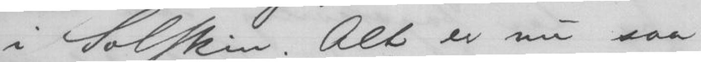i Solskin. Alt er nu saa
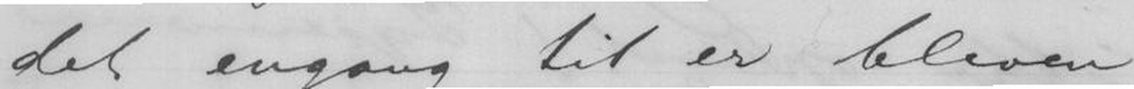det engang til er bleven
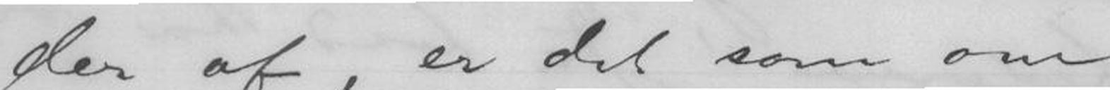der af, er det som om
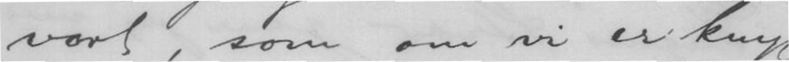vort, som om vi er knyt-
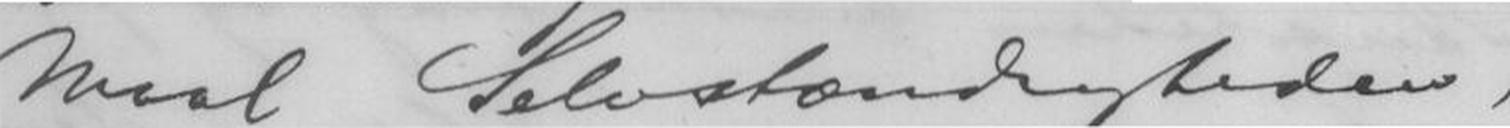Maal Selvstændigheden,
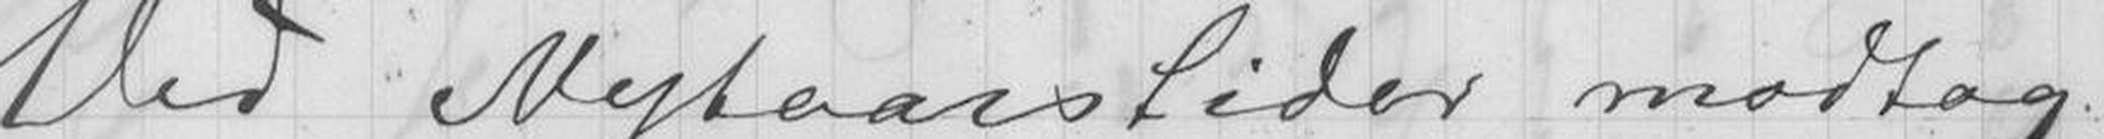Ved Nytaarstider modtog
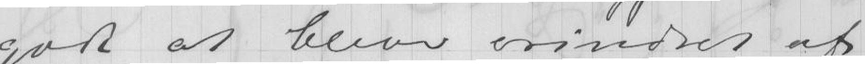godt at blive erindret af
